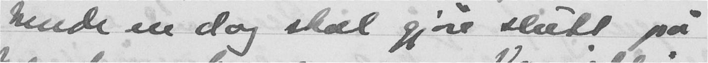hende en dag skal gjøre slutt på,
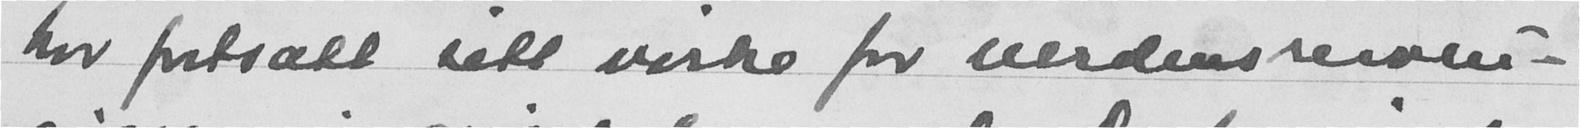har fortsatt sitt virke for verdensrevolu-
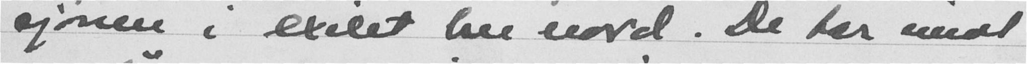sjonen i exilet her nord. De tar minst
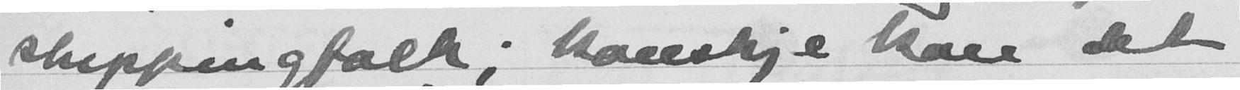skippingfolk; kanskje kan det
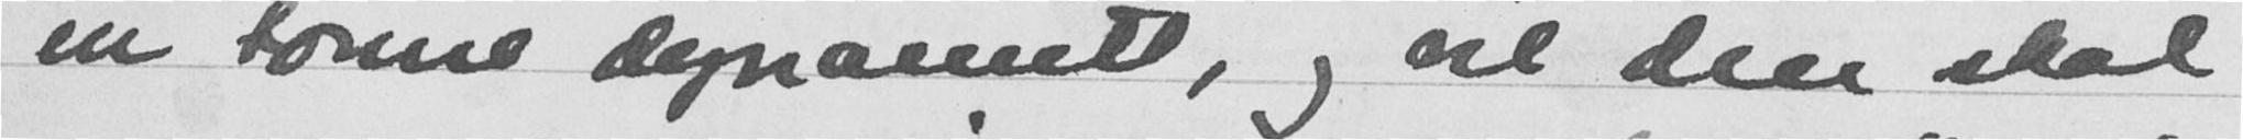en tønne dynamitt, og vil den skal
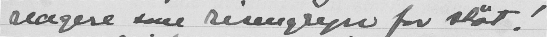reagere som risengryn for støt.
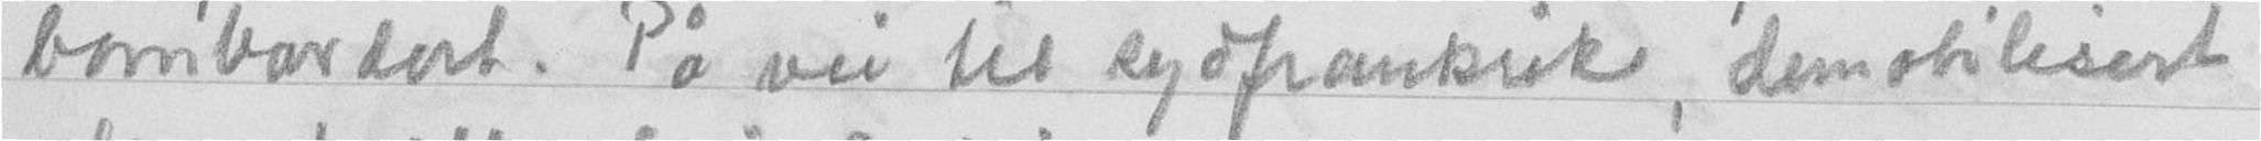bombardert. På vei til sydfrankrik. demobilisert
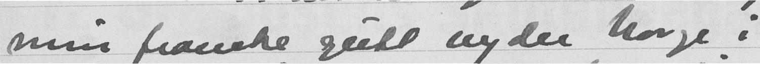min franske gutt nyder Norge i
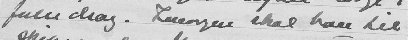fulle drag. Imorgen skal han til
